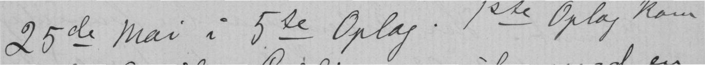25de mai i 5te Oplag. 1ste Oplag kom
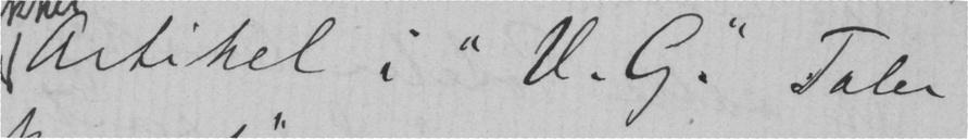Artikel i "V. G." Taler
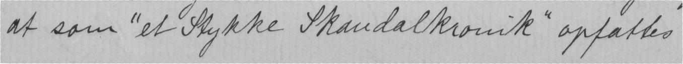at som "et Stykke Skandalkronik" opfattes
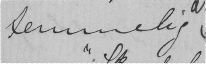temmelig
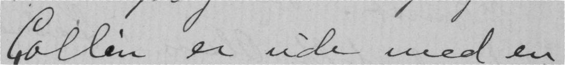Collin er ude med en
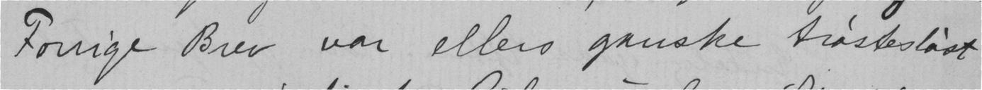Forrige Brev var ellers ganske trøstesløst
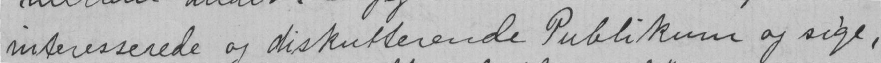interesserede og diskutterende Publikum og sige,
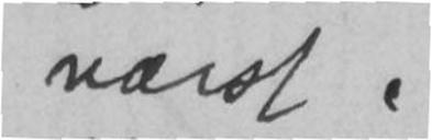værst.
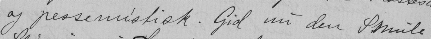og pessemistisk. Gid nu den Smule
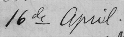16de April.
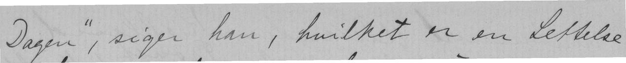Dagen", siger han, hvilket er en Lettelse
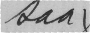saa
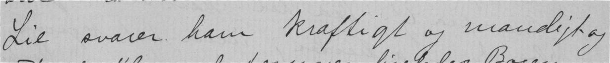Lie svarer ham kraftigt og mandigt og
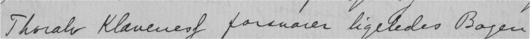Thoralv Klaveness forsvarer ligeledes Bogen
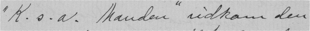"K. s. a. Manden" udkom den
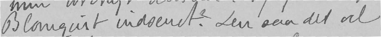Blomquist indsendt? Du saa det vel
